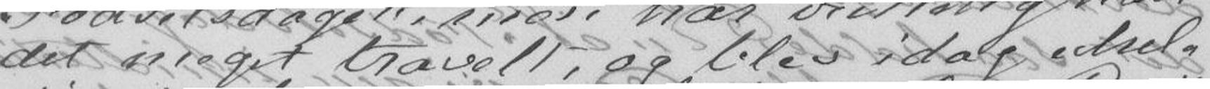det meget travelt, og blev idag uhel-
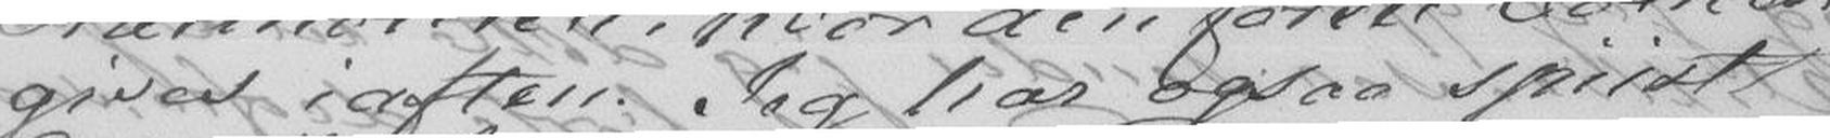gives iaften. Jeg har ogsaa spiist
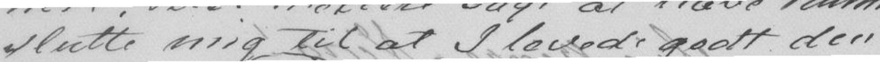slutte mig til at I levede godt den
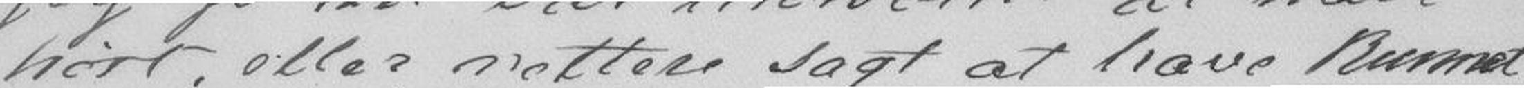hørt eller rettere sagt at have kunnet
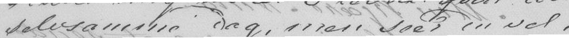selvsamme Dag, men seer Du vel,
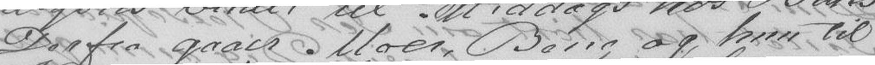Derfor gaaer Moer, Bene og hun til
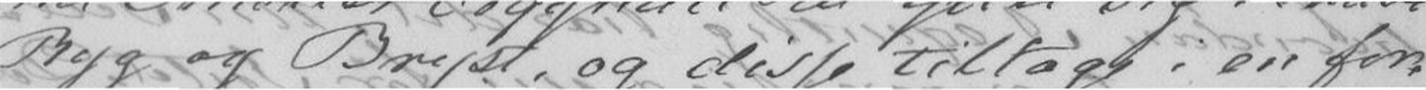Ryg og Bryst, og disse tiltage i en for-
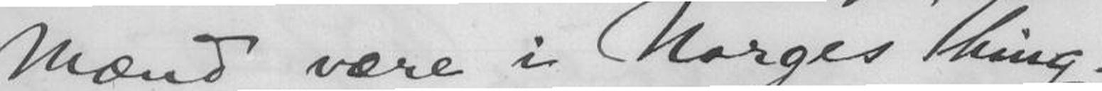Mænd være i Norges Thing-
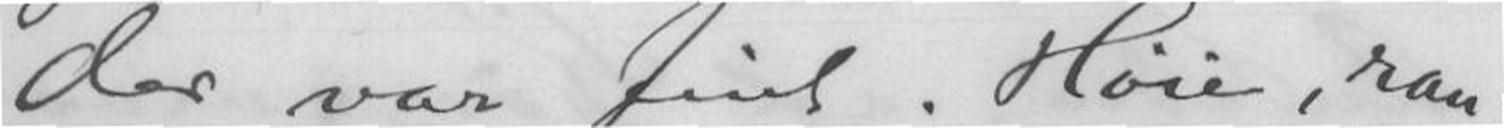der var fint. Høie, ran-
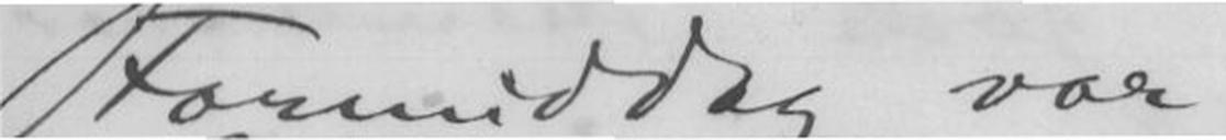Formiddag var
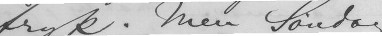tryk. Men Søndag
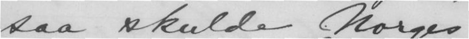saa skulde Norges
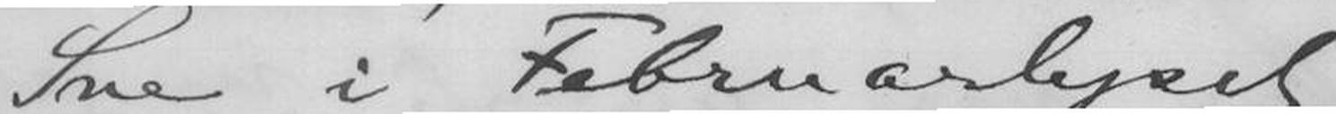Sne i Februarlyset, -
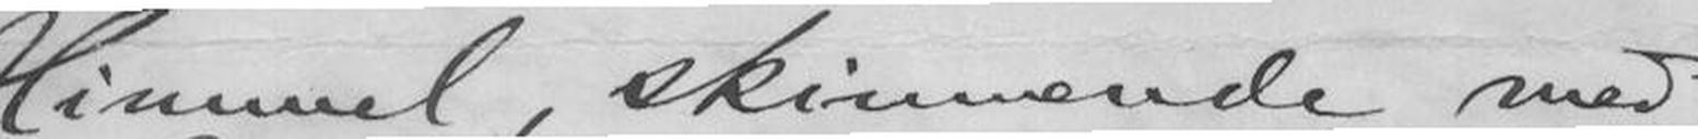Himmel, skinnende med
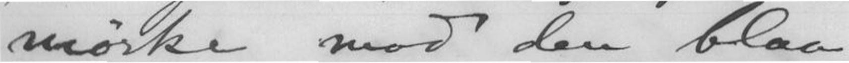mørke mod den blaa
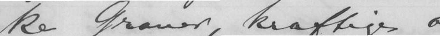ke Graner, kraftige og
